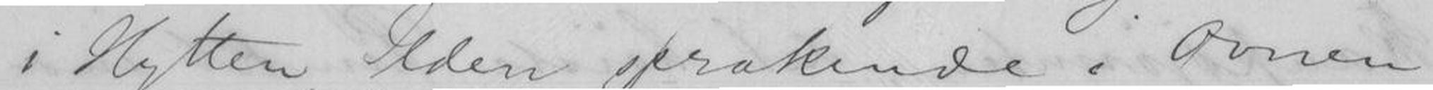i Hytten, Ilden sprakende i Ovnen
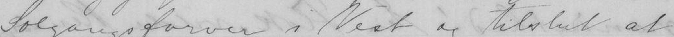Solgangsfarver i Vest og tilslut at
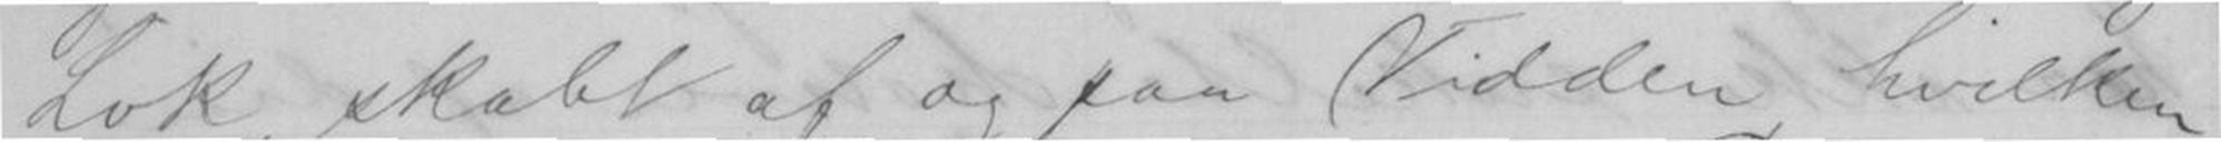Lok, skabt af og paa Vidden, hvilken
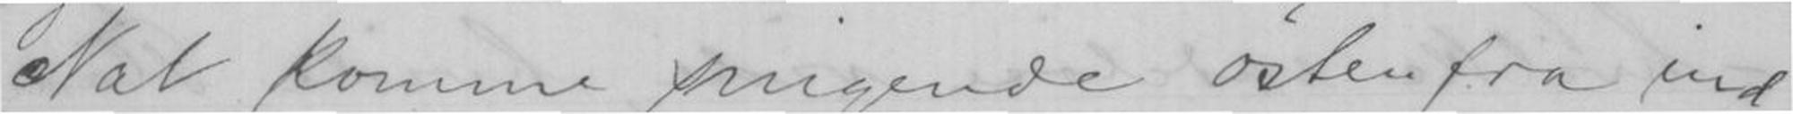Nat komme snigende østenfra ind-
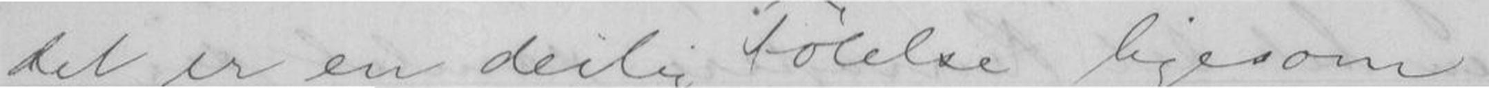det er en deilig Følelse ligesom
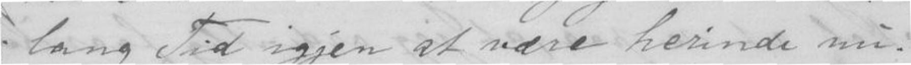lang Tid igjen at være herinde nu.
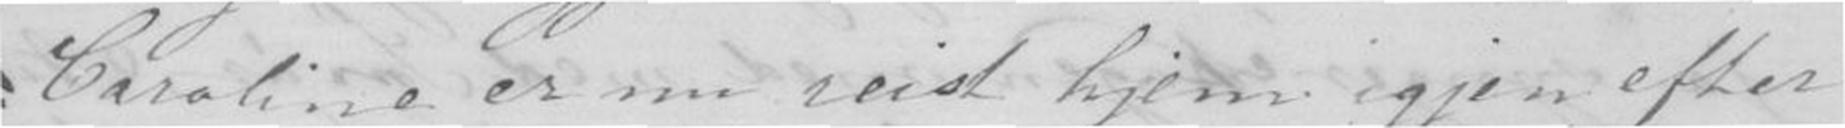Caroline er nu reist hjem igjen efter
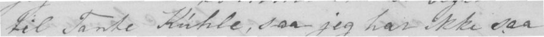til Tante Kühle, saa jeg har ikke saa
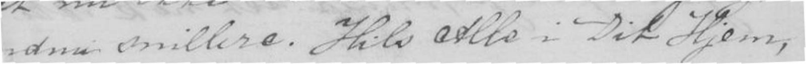ndnu snillere. Hils Alle i Dit Hjem,
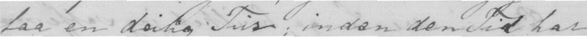faa en deilig Tur; inden den Tid har
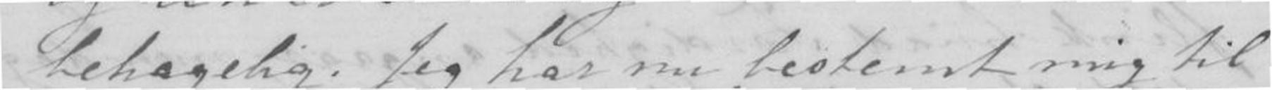behagelig. Jeg har nu bestemt mig til
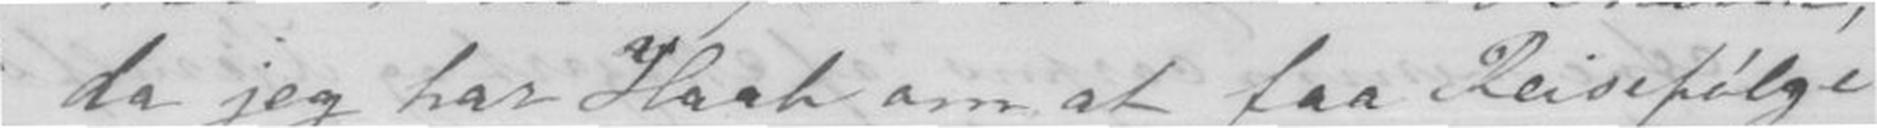da jeg har haab om at faa Reisefølge
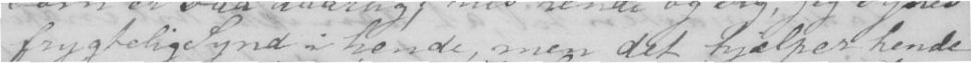frygtelig Synd i hende, men det hjælper hende
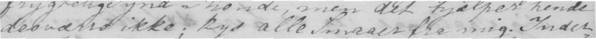desværre ikke; kys alle Smaaer fra mig. Inder-
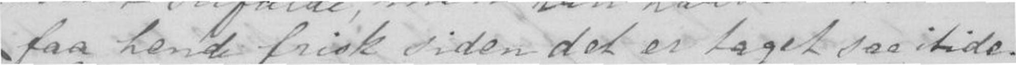faa hende frisk siden det er taget saa itide.
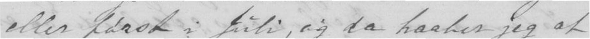eller først i Juli, og da haaber jeg at
